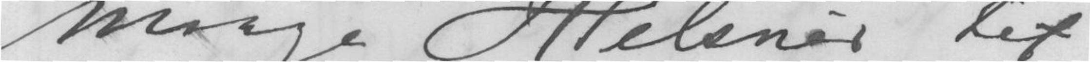mange Helsner til
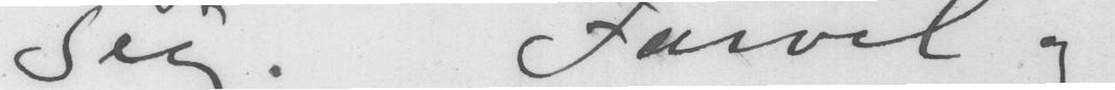seg. Farvel og
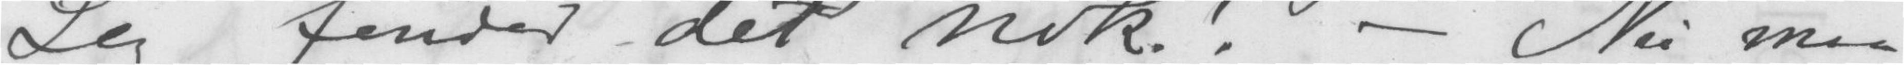Jeg finder det nok! - Nu maa
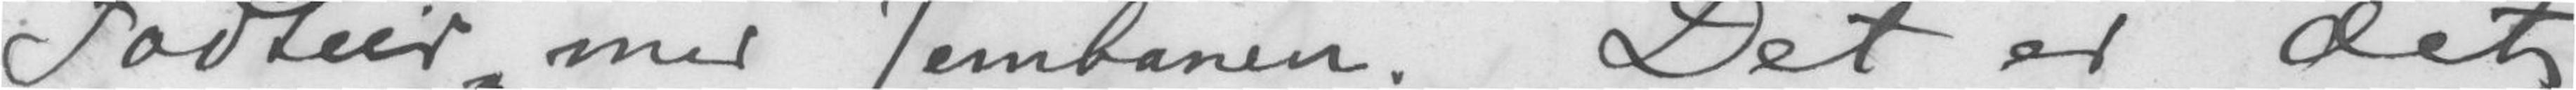Fodteir med Jernbanen. Det er det
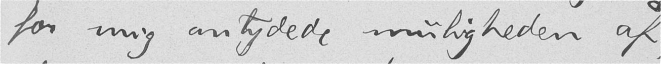for mig antydede muligheden af,
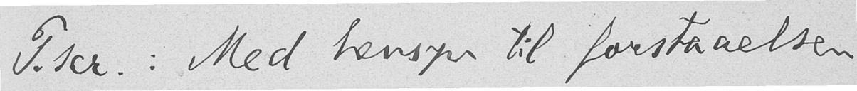P. scr.: Med hensyn til forstaaelsen
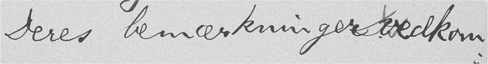Deres bemærkninger vedkom-
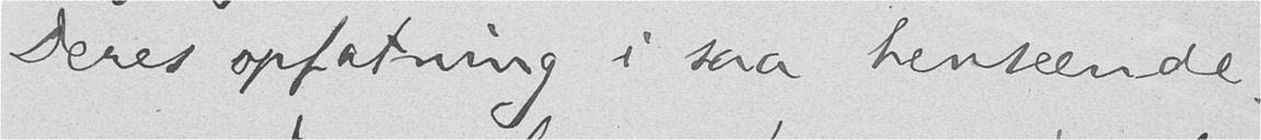Deres opfatning i saa henseende.
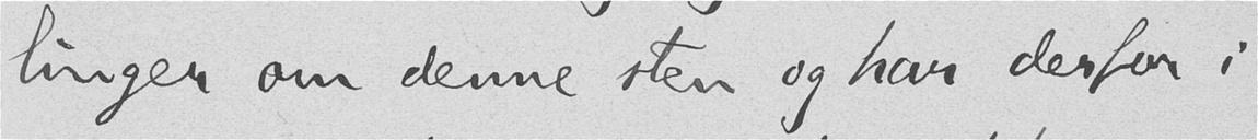linger om denne sten og har derfor i
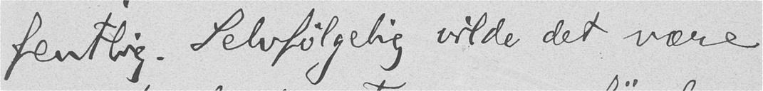fentlig. Selvfølgelig vilde det være
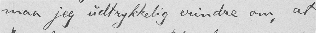maa jeg udtrykkelig erindre om, at
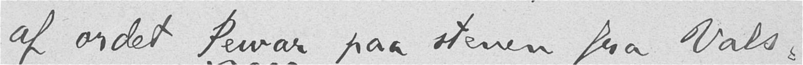af ordet Þewar paa stenen fra Vals-
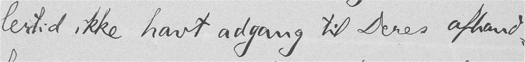lertid ikke havt adgang til Deres afhand-
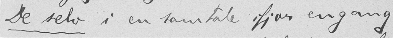De selv i en samtale ifjor en gang
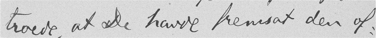troede, at De havde fremsat den of-
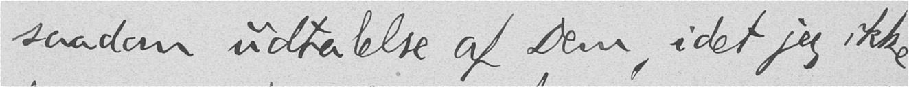saadan udtalelse af Dem, idet jeg ikke
